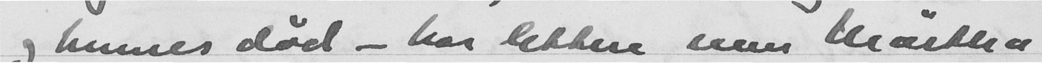og hennes død - har lettere enn Märtha
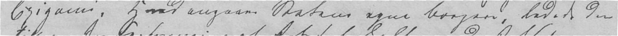Epigami. Hvad angaaer Statens egne Borgere, ledede den
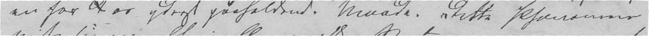en for d os yderst paafaldende Maade. Dette Phænomen
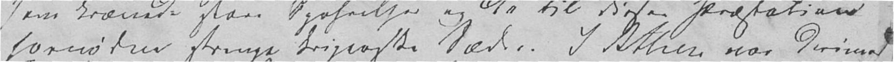fornødne strænge krigerske Sæder. I Athen var derimod
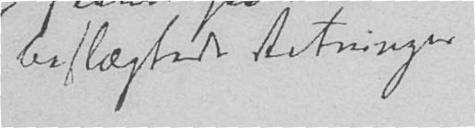beslægtede Retninger
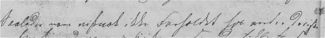Saaledes vare vistnok ikke Forholdet hos andre doriske
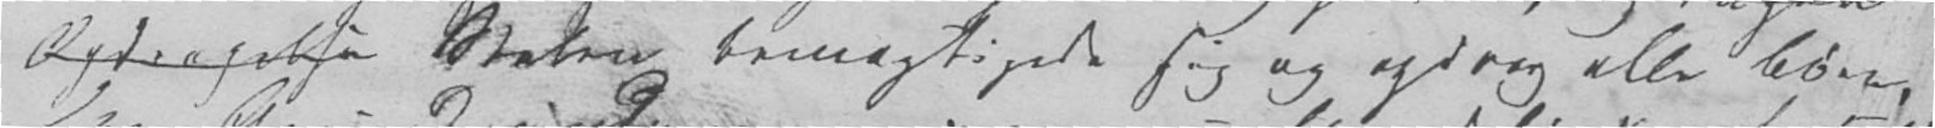Opdragelse Staten bemægtigede sig og opdrog alle Børn,
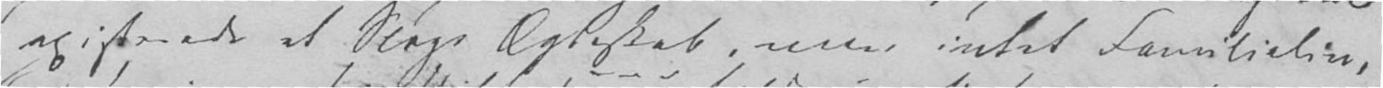existerede et Slags Ægteskab, men intet Familieliv,
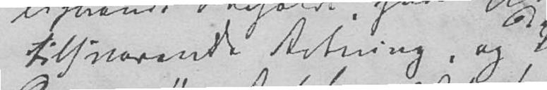tilsvarende Retning, og
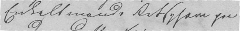Enkeltmands Retssphære paa
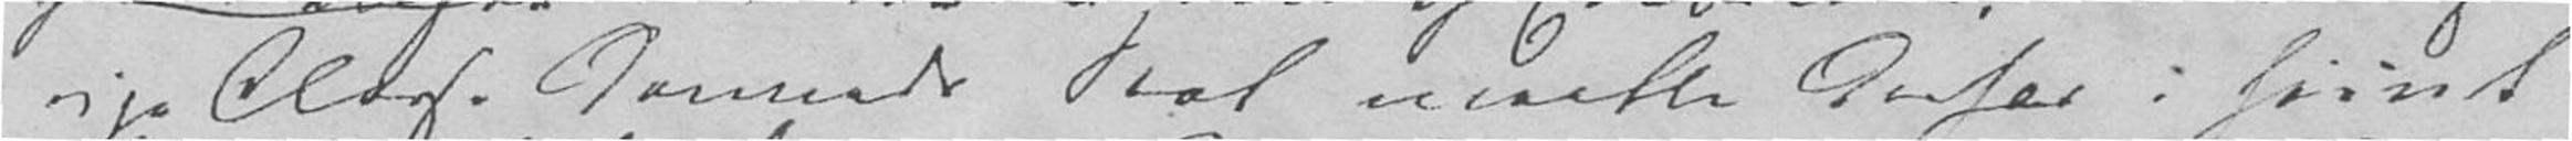rige Classe dannede Stat maatte derfor i hiint
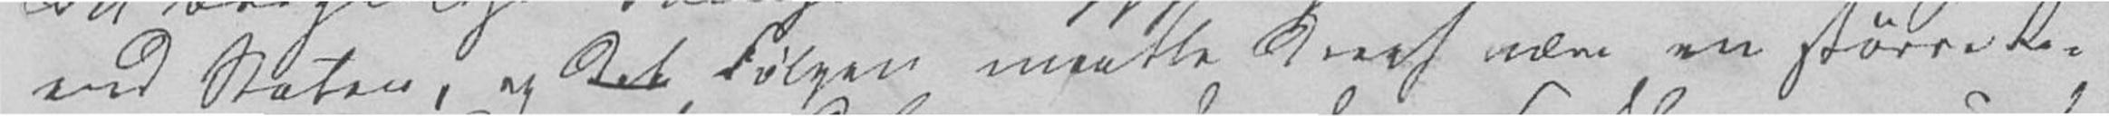end Staten, og det Følgen maatte deraf være en større Re-
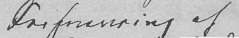Forverring af
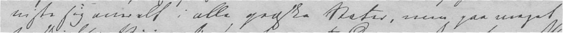viste sig overalt i alle græske Stater, men paa noget
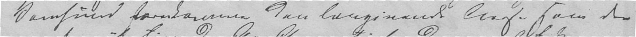Samfund forekomme den lovgivende Classe som den
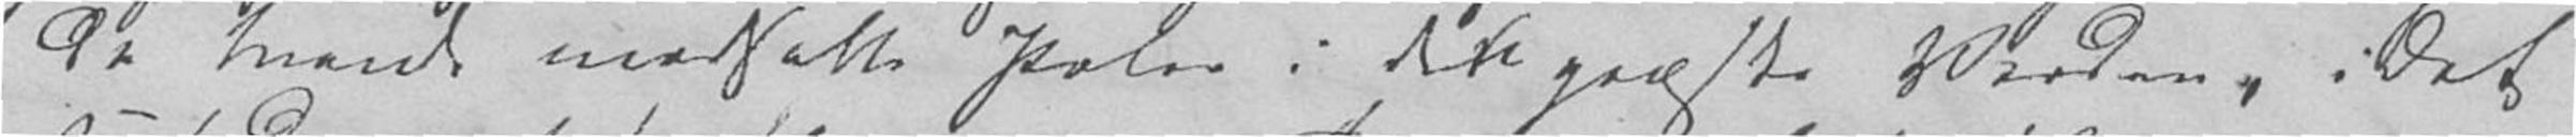de tvende modsatte Poler i den græske Verden, i det
